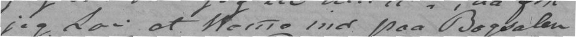jeg Lov at ko`me ind paa Bogsalen
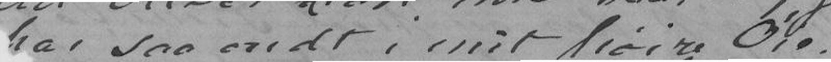har saa ondt i mit høire Øie
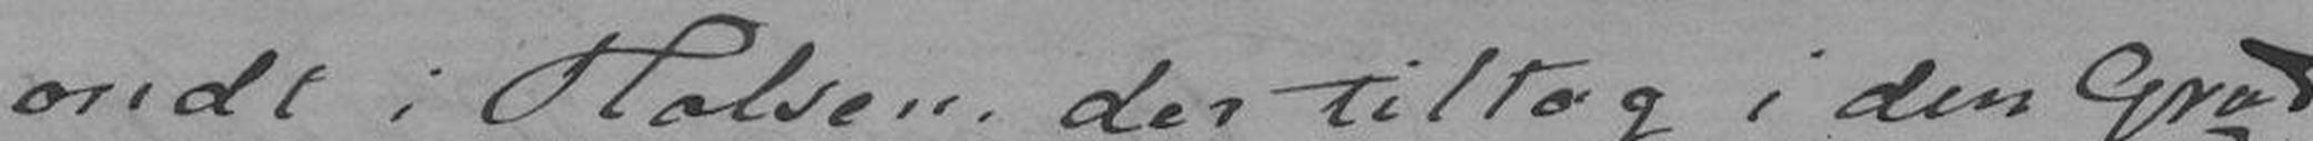ondt i Halsen, der tiltog i den Grad
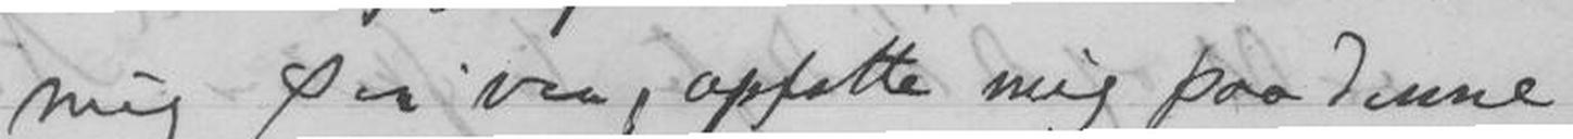mig sin ven, opfatte mig paa denne
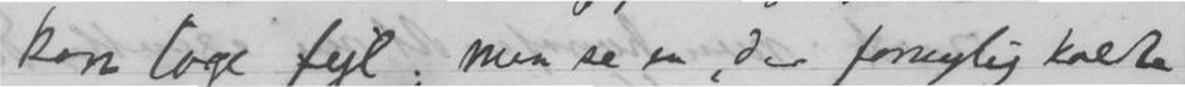kan tage fejl, men se en, der fornylig, kalte
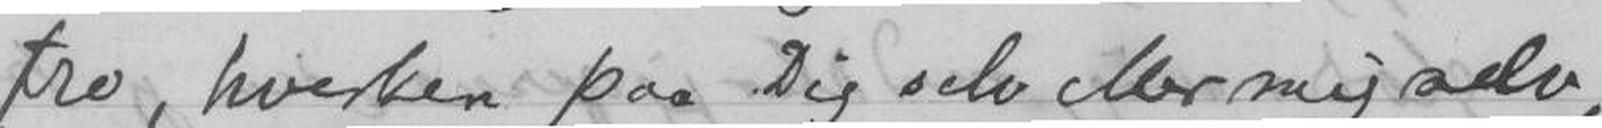tro, hverken paa Dig selv eller mig selv,
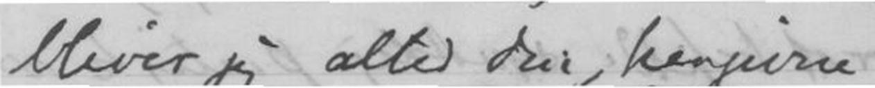bliver jeg altid din hengivne
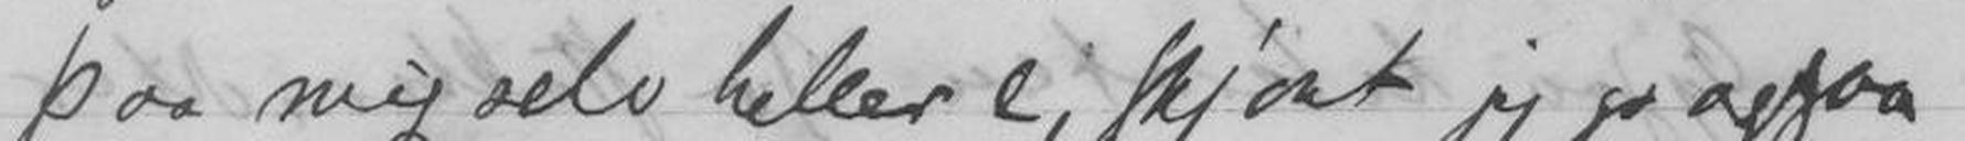paa mig selv heller ej skjønt jeg jo ogsaa
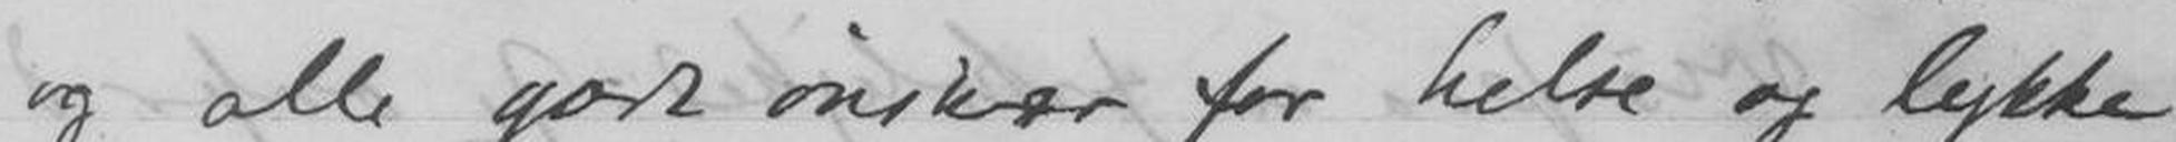og alle gode ønsker for helse og lykke
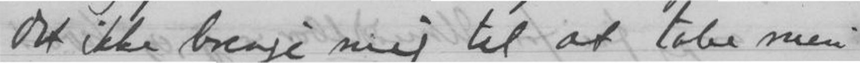det ikke bringe mig til at tabe min
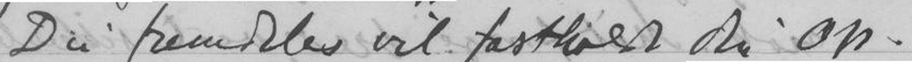Du fremdeles vil fasthoet din Op-
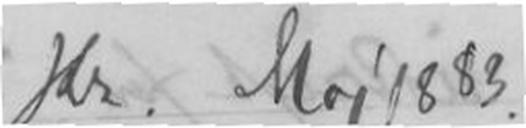sdr Maj 1883.
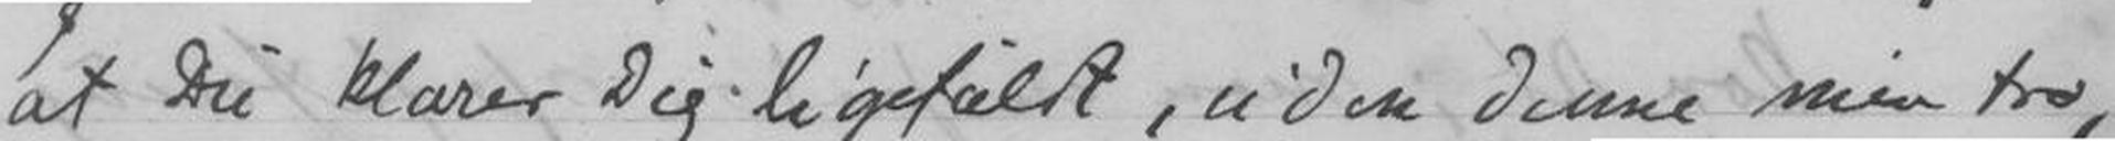at Du klarer Dig ligefuldt, uden denne men tro,
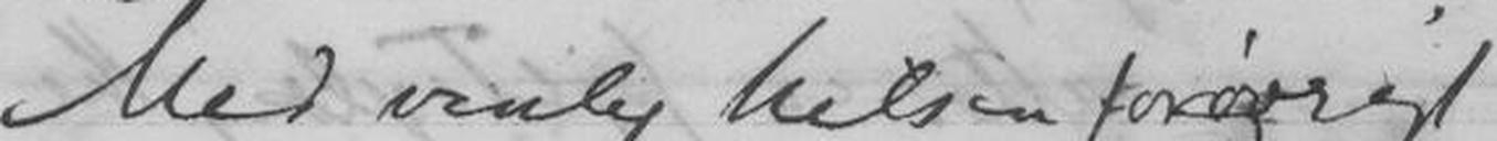Med venlig hilsen forøvrigt
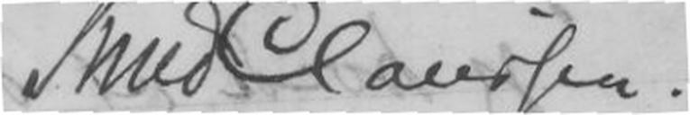Knud Claussen
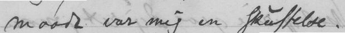maade var mig en skuffelse.
